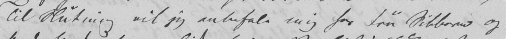Til Slutning vil jeg anbefale mig hos Fru Sibbern og
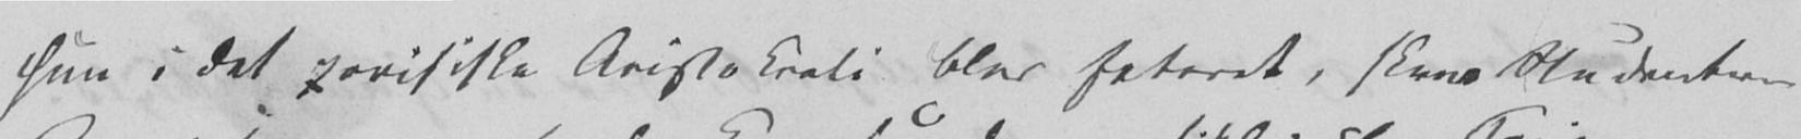hun i det parisiske Aristokrati blev feteret, skrev Studenterne
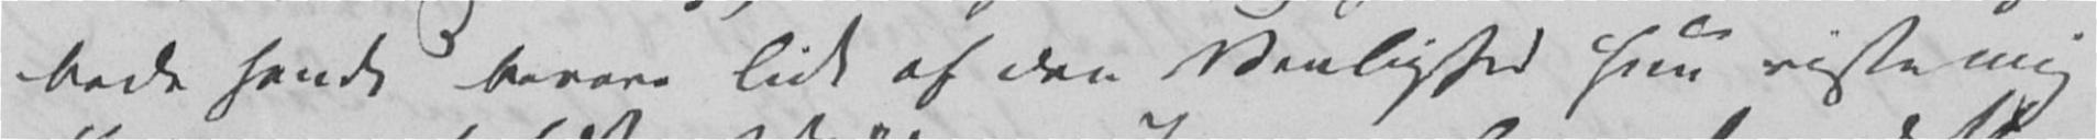bede hende bevare lidt af den Venlighed hun viste mig
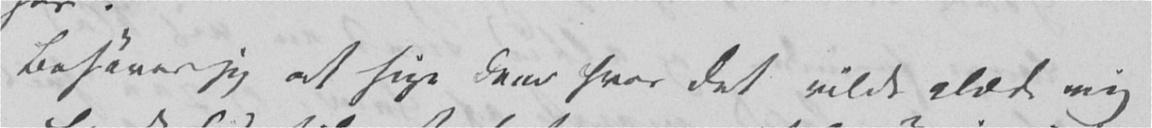Behøver jeg at sige Dem hvor det vilde glæde mig
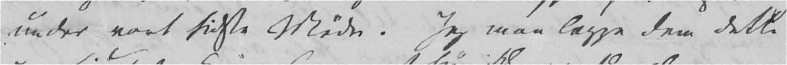under vort sidste Møde. Jeg maa lægge Dem dette
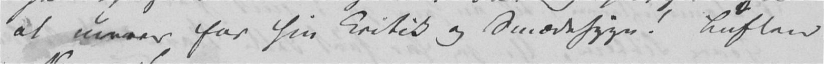at cureres for sin Kritik og Smædesyge! Luften
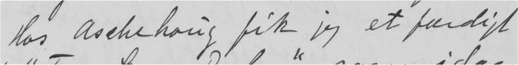Hos Aschehoug fik jeg et færdigt
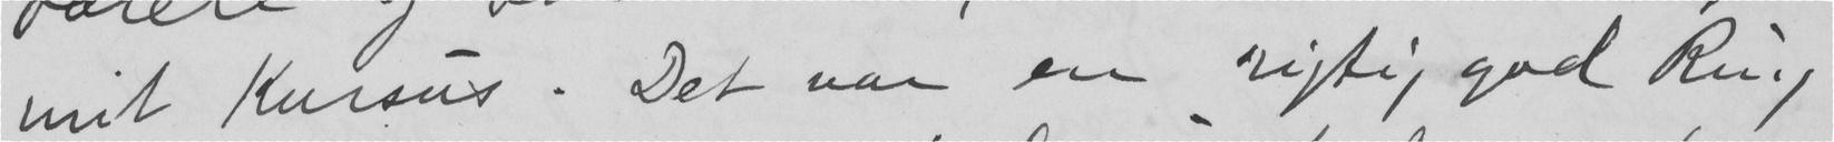mit Kursus. Det var en rigtig god Ring
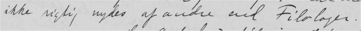ikke rigtig nydes af andre end Filologer.
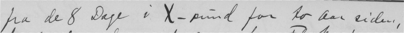fra de 8 Dage i X-sund for to Aar siden,
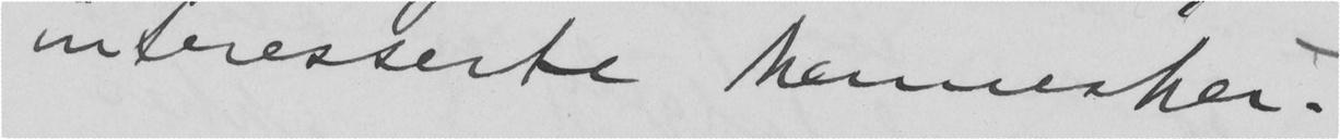interesserte Mennesker.
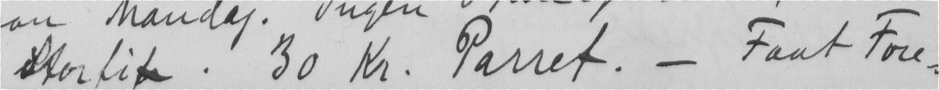storfif. 30 Kr. Parret. - Faat Fore-
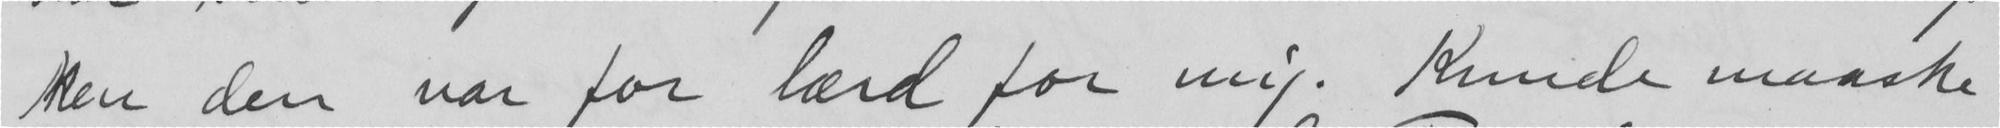Men den var for lærd for mig. Kunde maaske
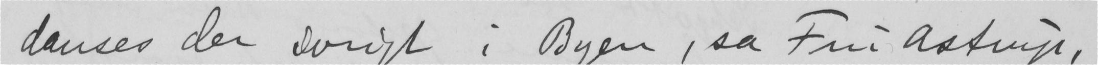danses der øvrigt i Byen, sa Fru Astrup,
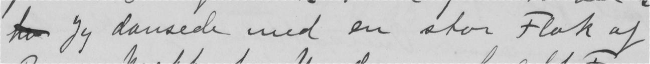Jeg dansede med en stor Flok af
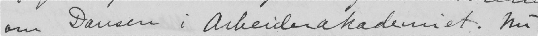om Dansen i Arbeiderakademiet. Nu
